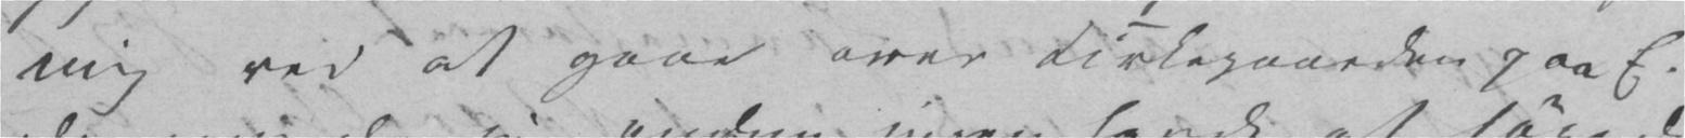mig ved at gaae over Kirkegaarden paa E.
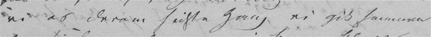vi os derom sidste Gang vi gik sammen
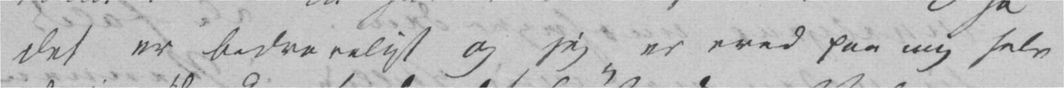det er bedrøveligt og jeg er vred paa mig selv
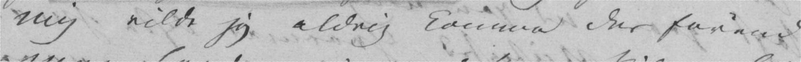mig vilde jeg aldrig komme der førend
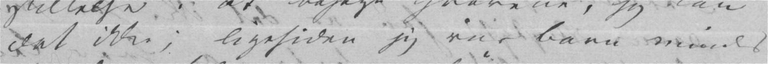det ikke; ligesiden jeg var Barn mindes
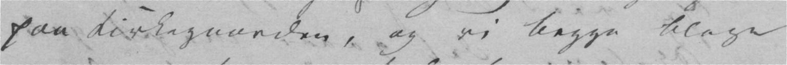paa Kirkegaarden, og vi begge blege
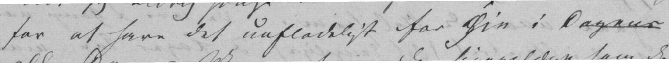for at have det uafladeligt for Øie i Dagens
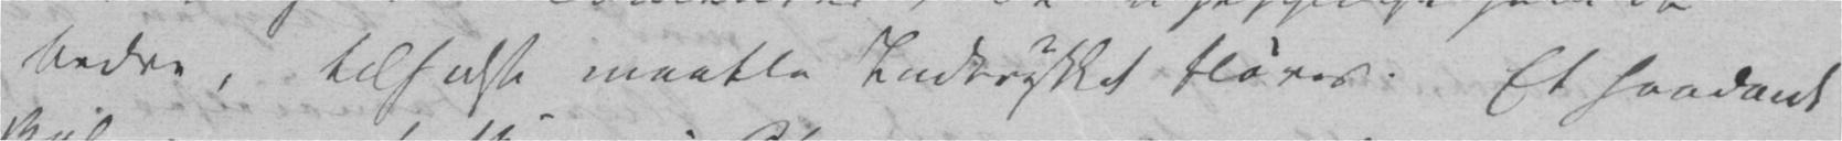bedre, tilsidst maatte Indtrykket sløves. Et saadant
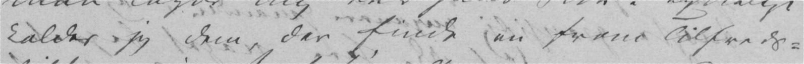kalder jeg dem, der finde en from Tilfreds-
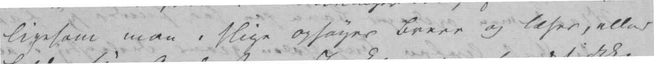ligesom man i slige opsøger Breve og læser, eller
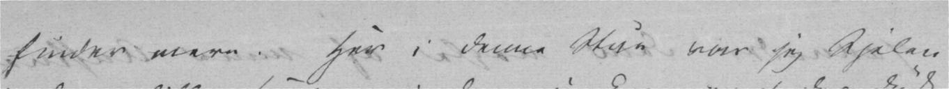finder mere. Her i denne Stue var jeg Sjelen
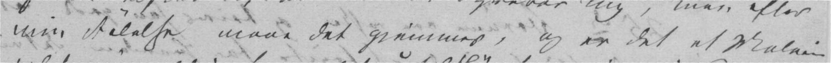min Følelse maae det gjemmes, og er det et Malerie
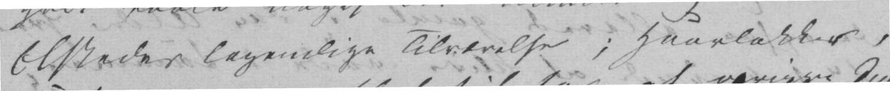Elskedes legemlige Tilværelse; Haarlokker,
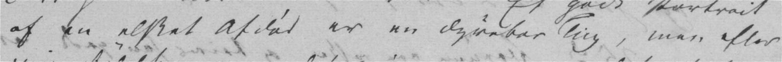af en elsket Afdød er en dyrebar Ting, men efter
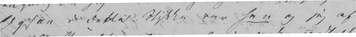Ogsaa i dette Stykke var han og jeg af
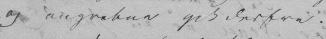og angrebne gik derfra.
